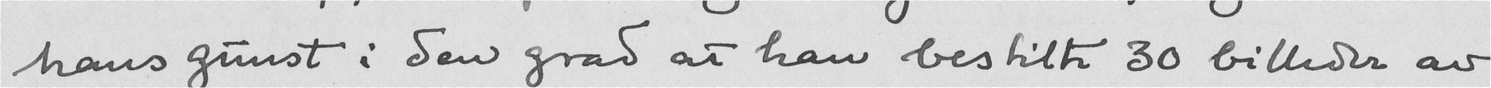hans gunst i den grad at han bestilte 30 billeder av
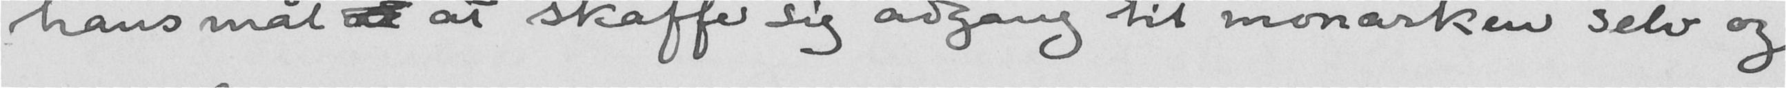hans mål at at skaffe sig adgang til monarken selv og
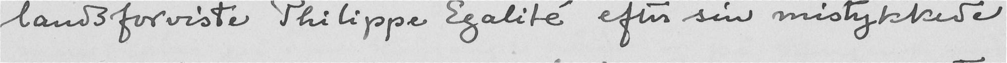landsforviste Philippe Egalité efter sin mislykkede
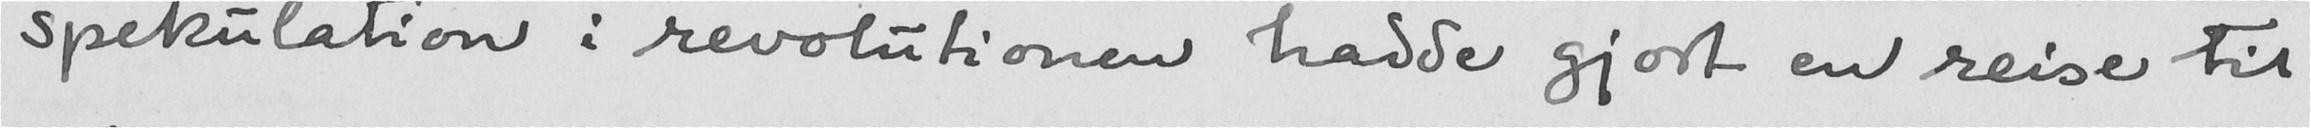spekulation i revolutionen hadde gjort en reise til
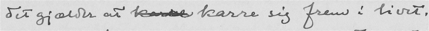det gjælder at kare karre sig frem i livet.
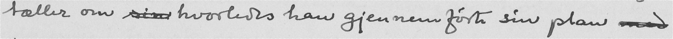tæller om sin hvorledes han gjennemførte sin plan med
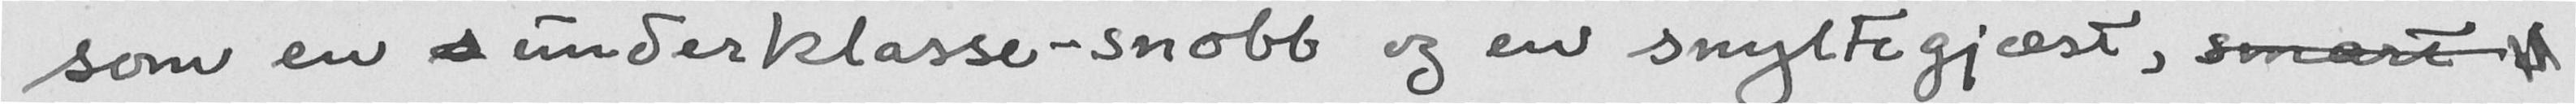som en underklasse-snobb og en snyltegjæst, smart
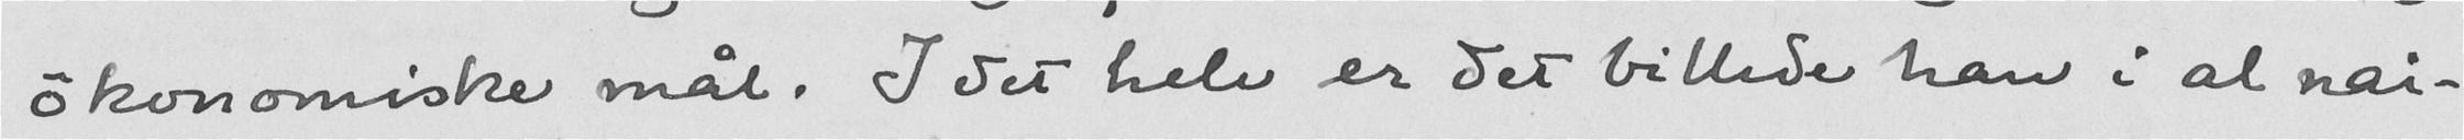økonomiske mål. I det hele er det billede han i al nai-
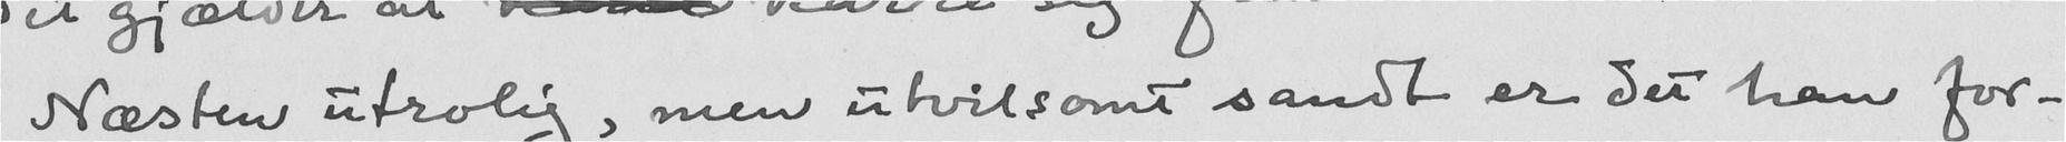Næsten utrolig, men utvilsomt sandt er det han for-
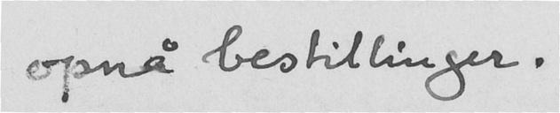opnå bestillinger.
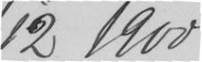30/12 1900
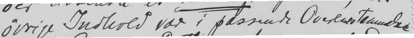øvrige Indhold var i passende Overenstemmelse
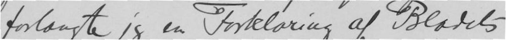forlangte jeg en Forklaring af Bladets
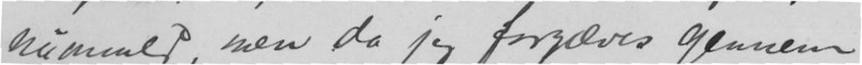nummeret, men da jeg forjæves gjennem
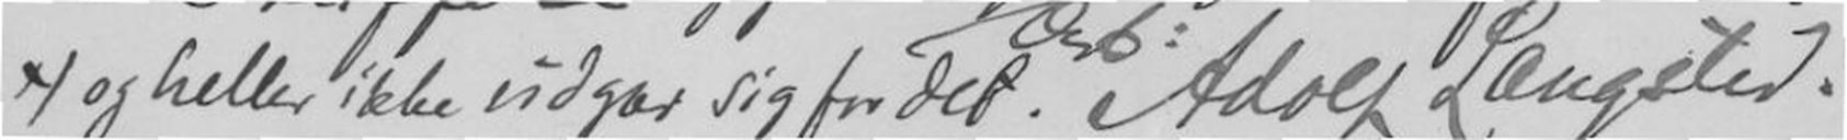*) og heller ikke udgaa sig for det. Adolf Langsted.
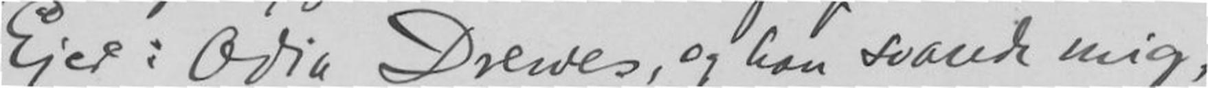Ejet: Odia Drewes, og han svarede mig,
